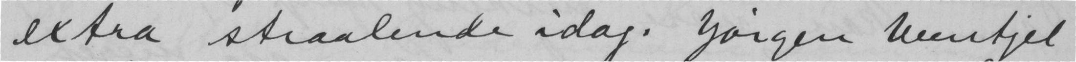extra straalende idag. Jørgen Wentjel
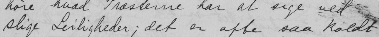slige Leiligheder; det er ofte saa koldt
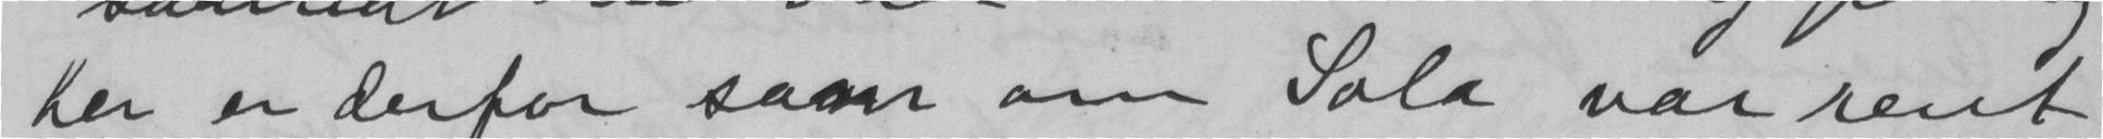her er derfor som om Sola var rent
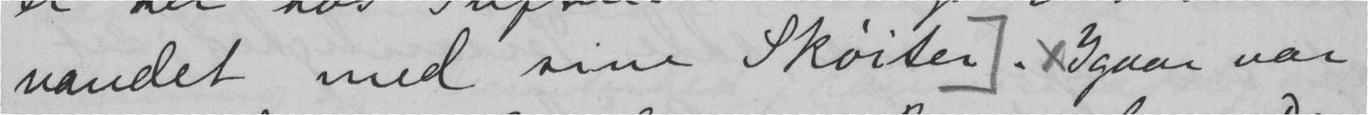vandet med sine Skøiter. x Igaar var
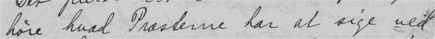høre hvad Præsterne har at sige ved
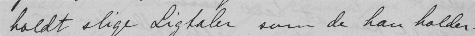holdt slige Ligtaler som de han holder
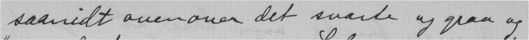saavidt ovenover det svarte og graa og
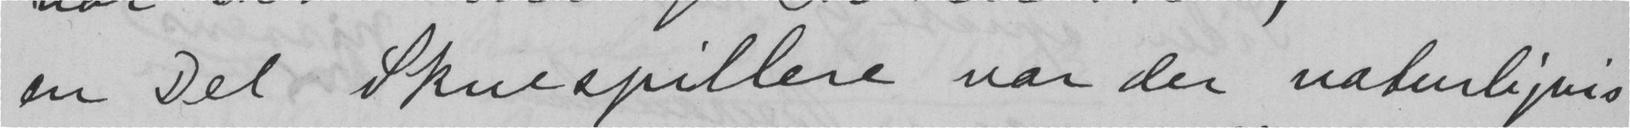en Del Skuespillere var der naturligvis
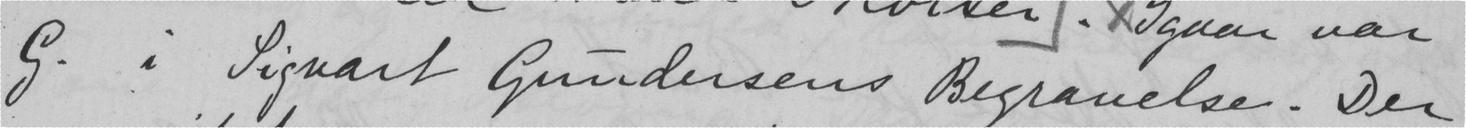G. i Sigvart Gundersens Begravelse. Der
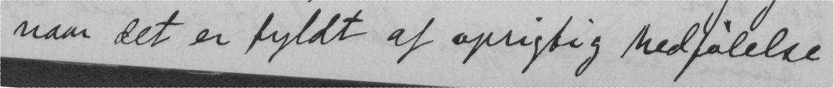naar det er fyldt af oprigtig medfølelse
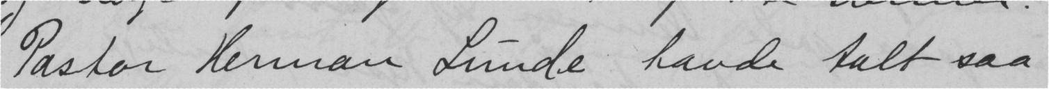Pastor Herman Lunde havde talt saa
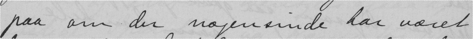paa om der nogensinde har været
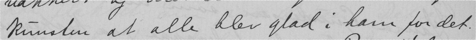kunsten at alle blev glad i ham for det.
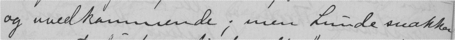og uvedkommende; men Lunde snakkere
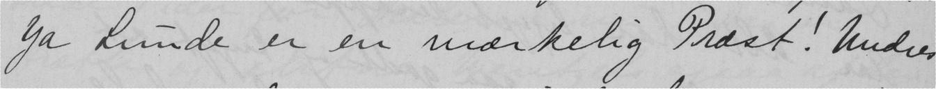Ja Lunde er en mærkelig Præst! Undres
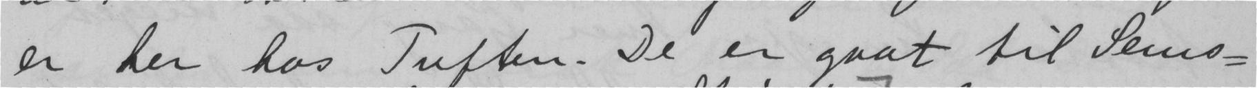er her hos Tuften. De er gaat til Sems-
